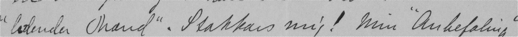"lidende Mand". Stakkars mig! Min "Anbefaling"
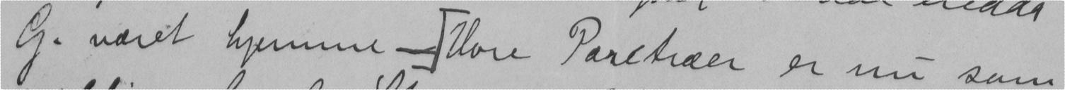G. været hjemme - Vore Pæretræer er nu som
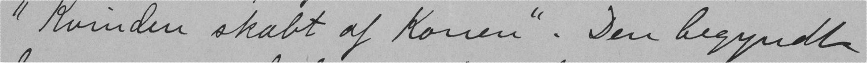"Kvinden skabt af Konen". Den begyndte
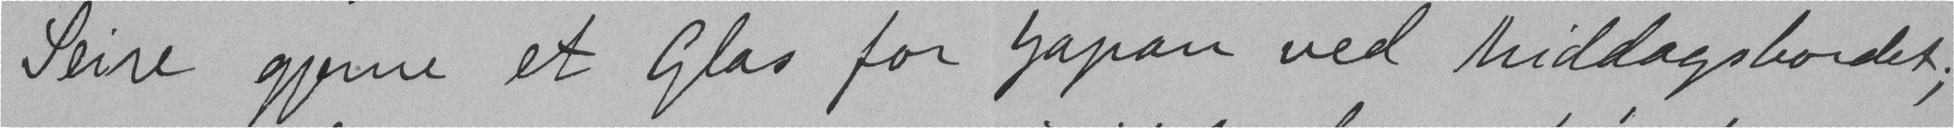Seire gjerne et Glas for Japan ved Middagsbordet;
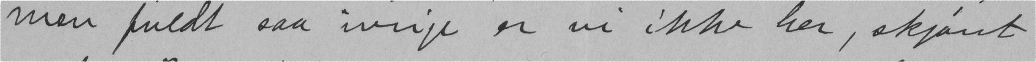men fuldt saa ivrige er vi ikke her, skjønt
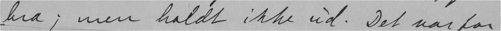bra; men holdt ikke ud. Det var for
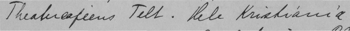Theatercaféens Telt. Hele Kristiania
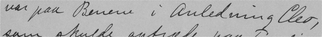var paa Benene i Anledning Cleo,
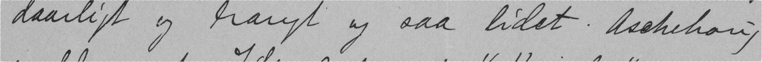daarligt og trangt og saa lidet. Aschehou,
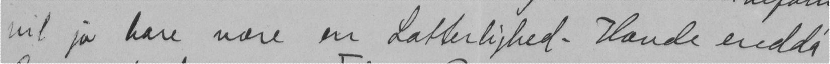vil jo bare være en Latterlighed. Havde endda
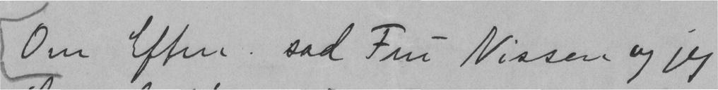Om Eftm. sad Fru Nissen og jeg
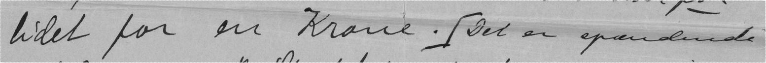lidet for en Krone. Det er spændende
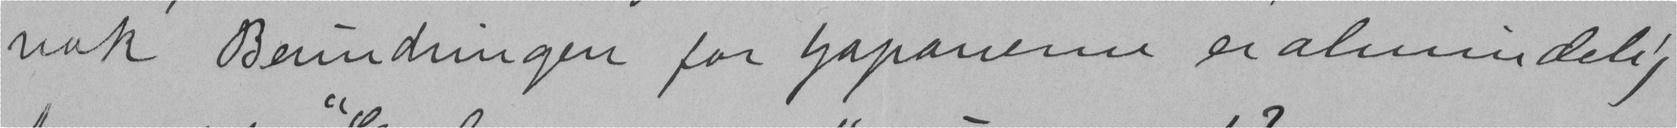nok Beundringen for Japanerne er almindelig
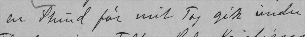en Stund før mit Tog gik undre
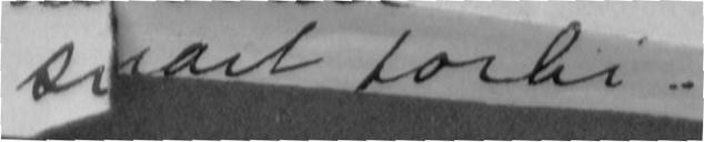snart forbi.
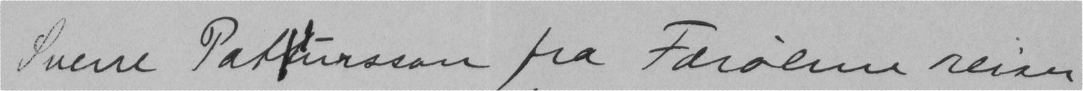Sverre Patursson fra Færøene reiser
